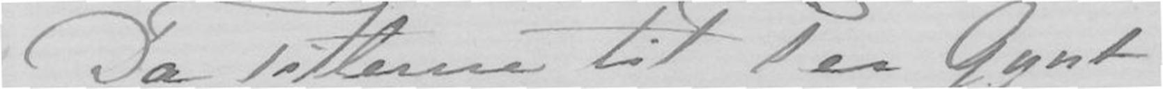Da Titlerne til Per Gynt
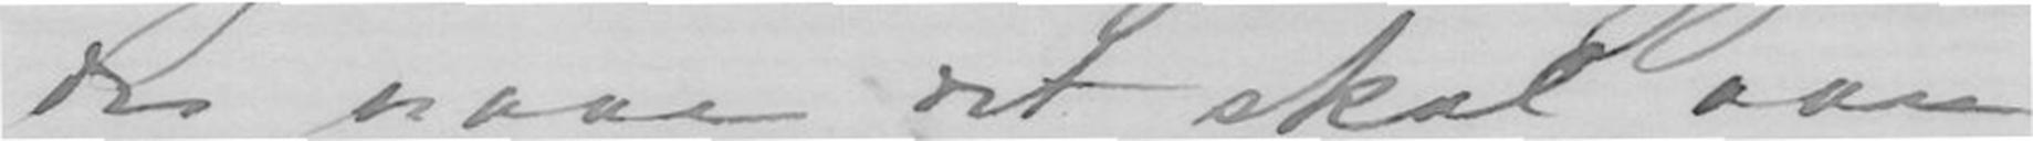des naar det skal være.
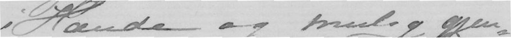i Hænde og mulig gjen-
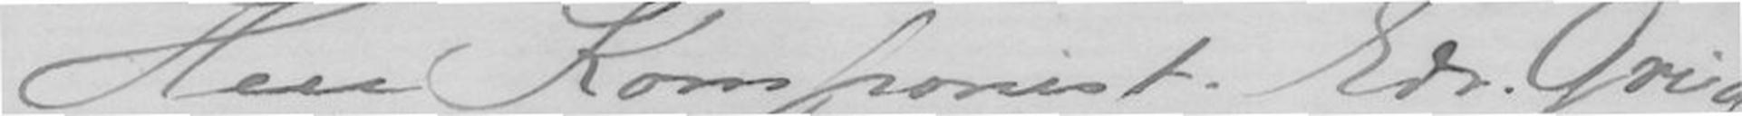Herr Komponist. Edv. Grieg.
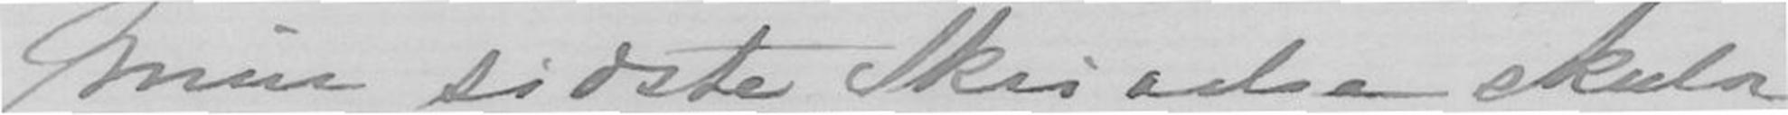min sidste Skrivelse skulde
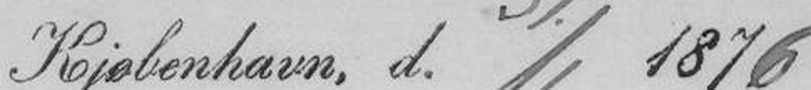Kjøbenhavn d. 21/1 1876
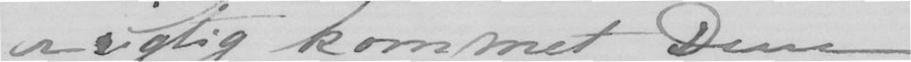er rigtig kommet Dem
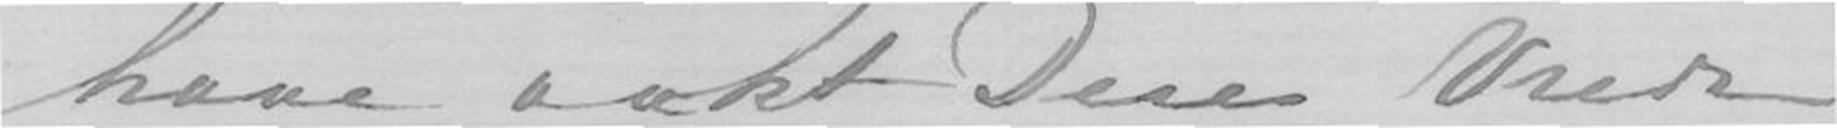have vakt Deres Vrede
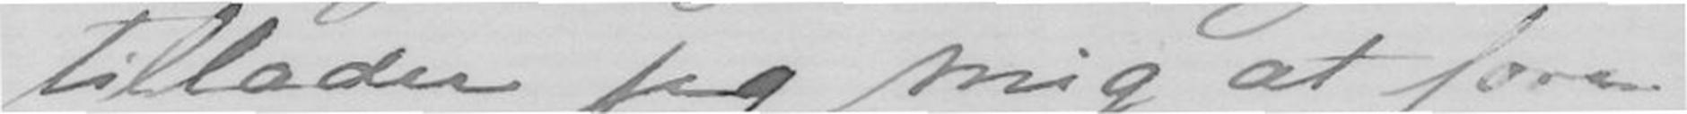tillader jeg mig at fore-
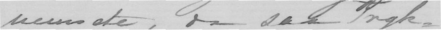nems etc, da saa Tryk-
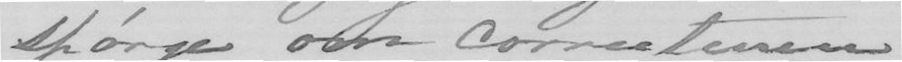spørge om Correcturen
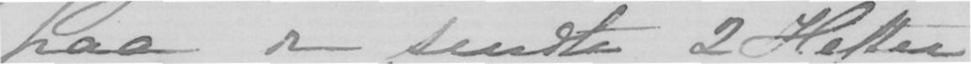paa de sendte 2 Hefter
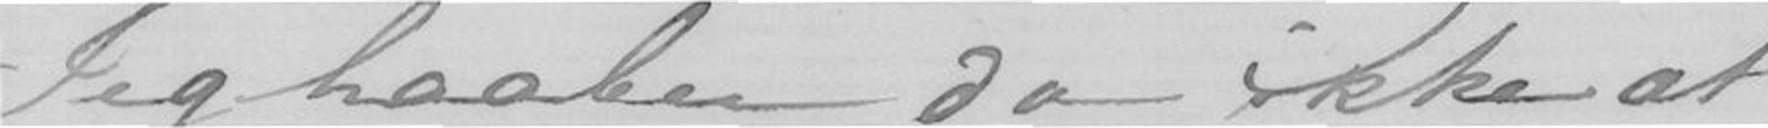Jeg haaber da ikke at
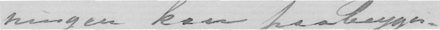ningen kan paabegyn-
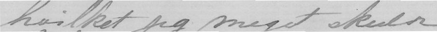hvilket jeg meget skulde
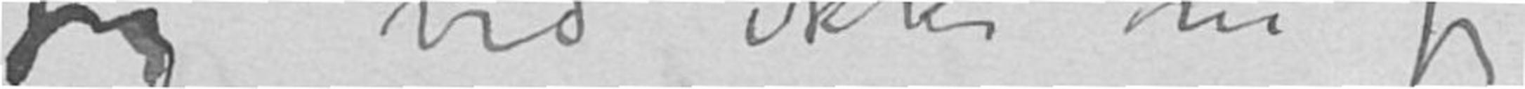Jeg ved ikke om jeg
